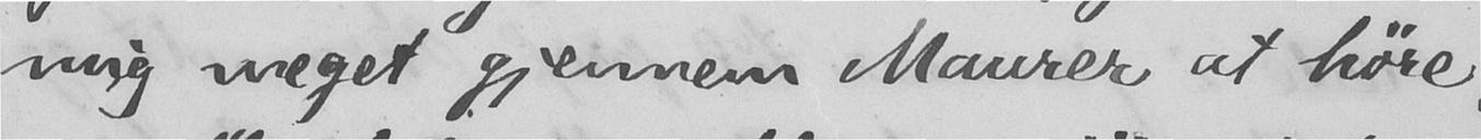mig meget gjennem Maurer at høre.
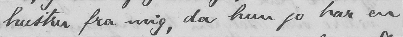hustru fra mig, da hun jo har en
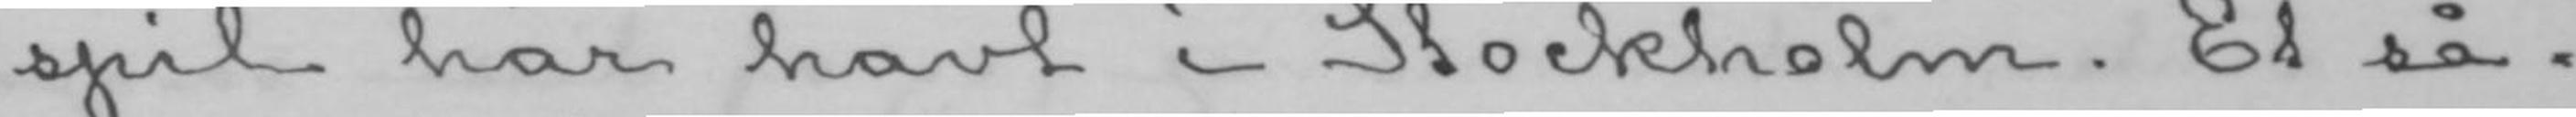spil har havt i Stockholm. Et så-
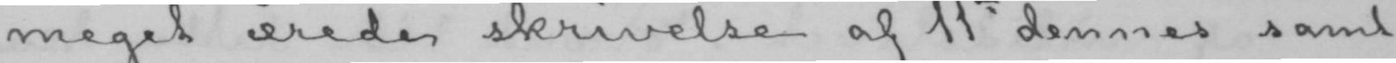meget ærede skrivelse af 11te dennes samt
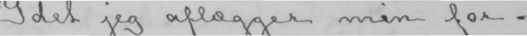Idet jeg aflægger min for-
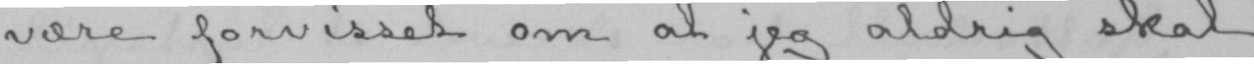være forvisset om at jeg aldrig skal
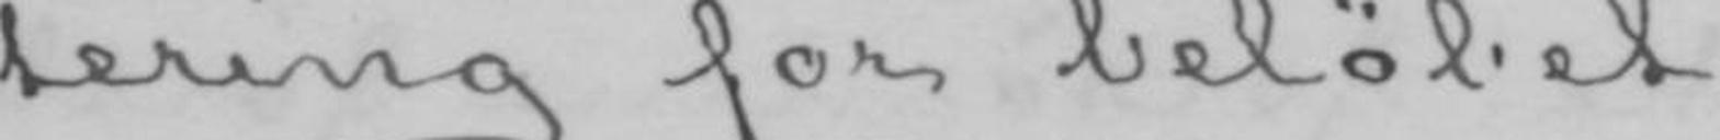tering for beløbet.
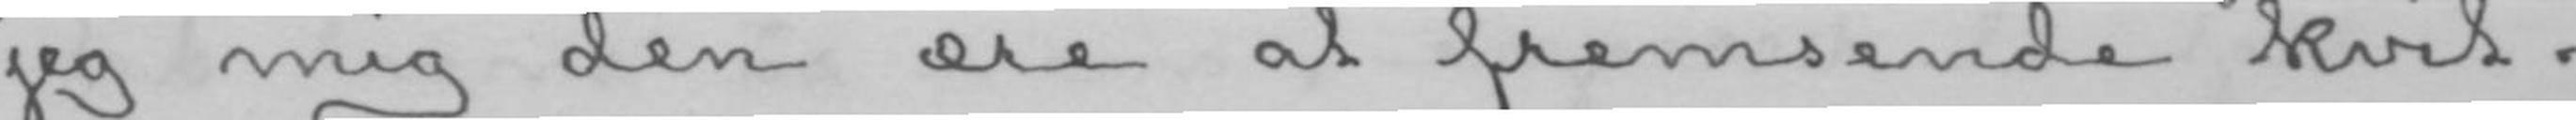jeg mig den ære at fremsende kvit-
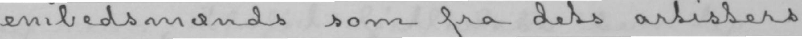embedsmænds som fra dets artisters
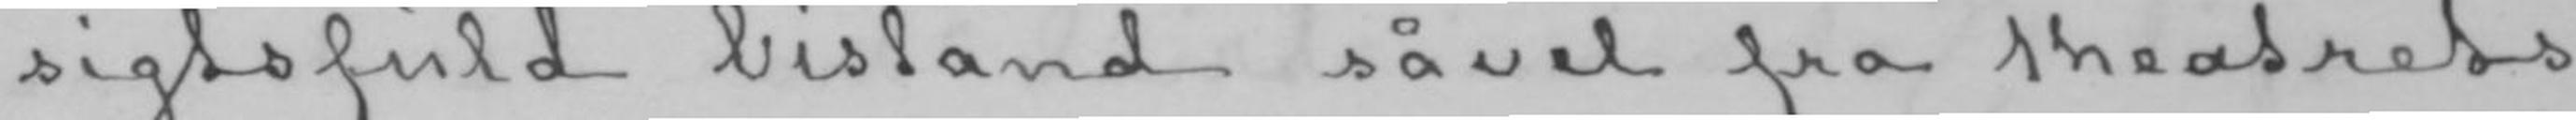sigtsfuld bistand såvel fra theatrets
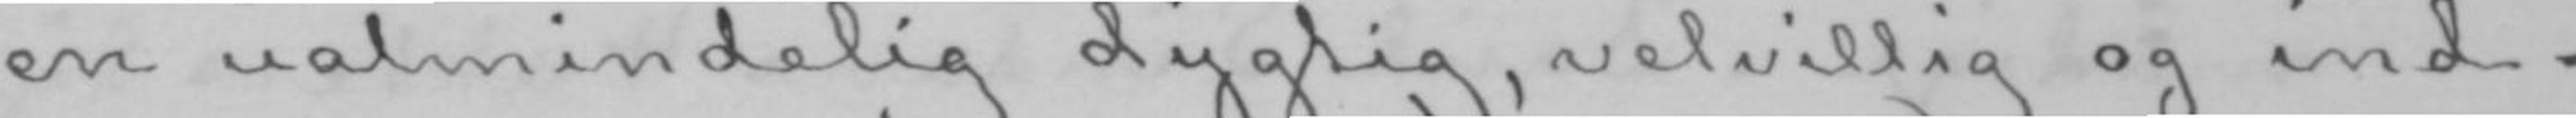en ualmindelig dygtig, velvillig og ind-
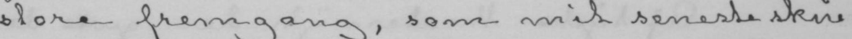store fremgang, som mit seneste skue-
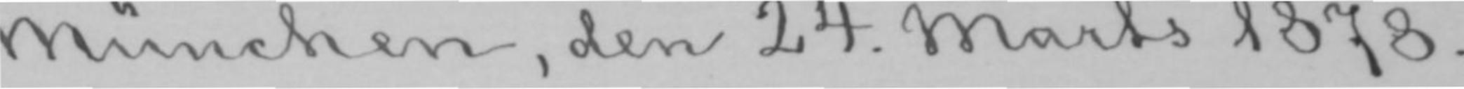München, den 24. Marts 1878.
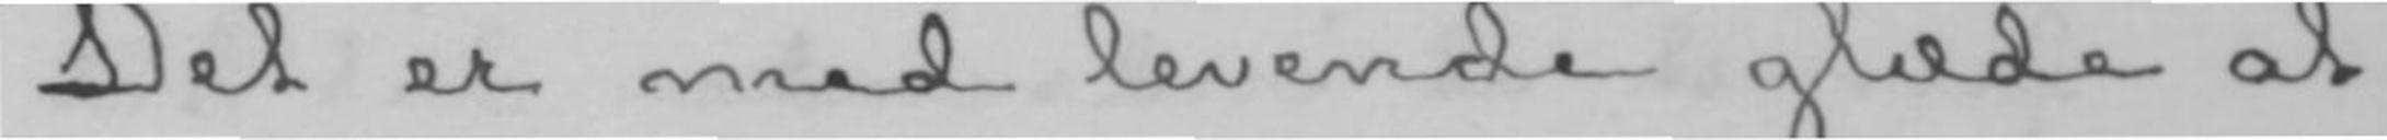Det er med levende glæde at
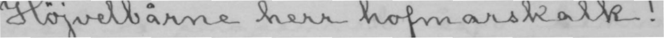Højvelbårne herr hofmarskalk!
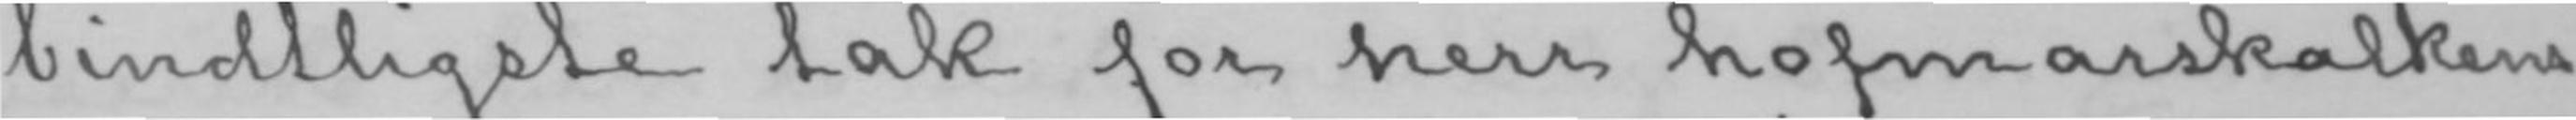bindtligste tak for herr hofmarskalkens
